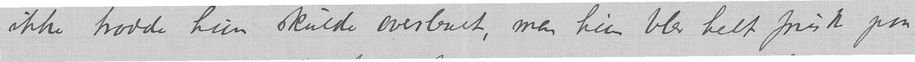ikke trodde hun skulde overlevet, men hun blev helt frisk paa
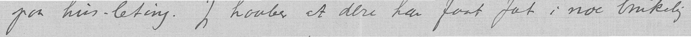paa hus-leting. Jeg haaber at dere har faat fat i noe brukelig
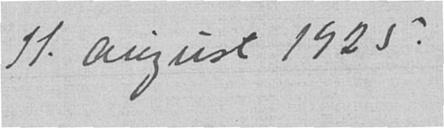11. august 1925.
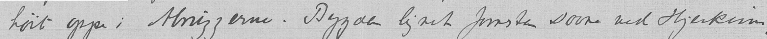høit oppe i Abruggerne. Bygden lignet forresten Dovre ved Hjerkinn,
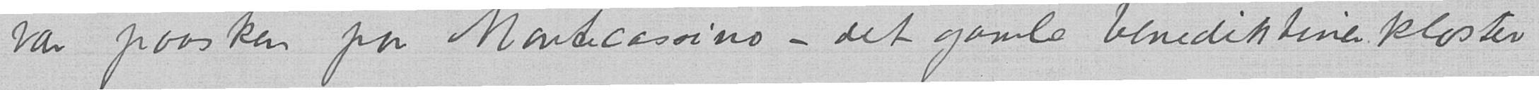var paasken paa Montecassino – det gamle benediktinerkloster
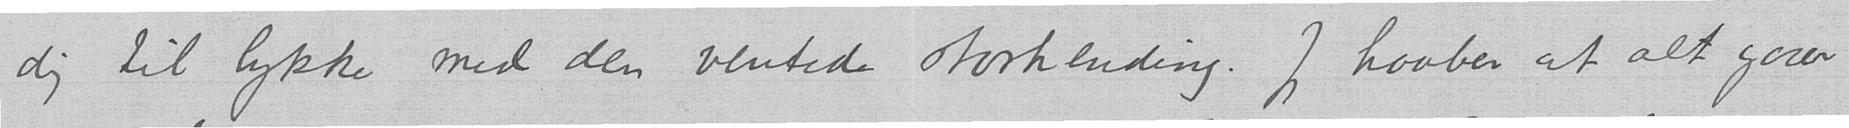dig til lykke med den ventede storhending. Jeg haaber at alt gaar
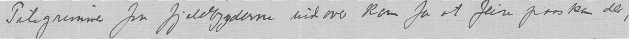Pilegrimmer fra fjellbygderne indover kom for at feire paasken der,
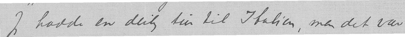Jeg hadde en deilig tur til Italien, men det var
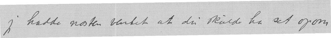jeg hadde næsten ventet at du skulde ha set opom
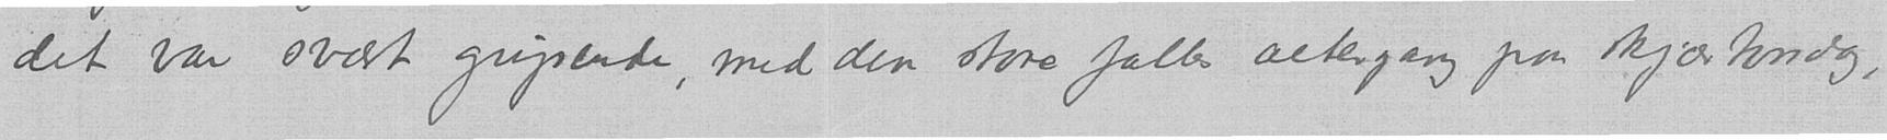det var svært gripende, med den store fælles altergang paa skjærtorsdag,
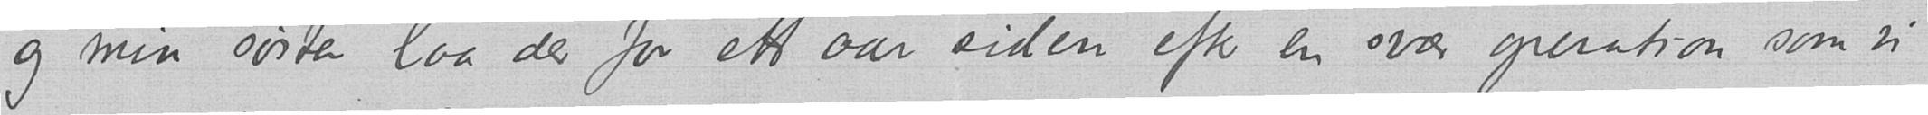og min søster laa der for ett aar siden efter en svær operation som vi
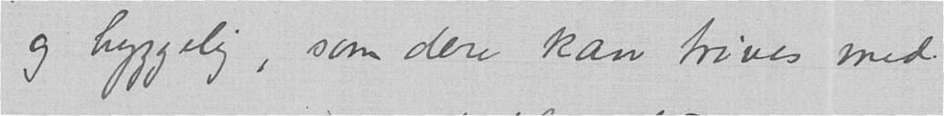og hyggelig, som dere kan trives med
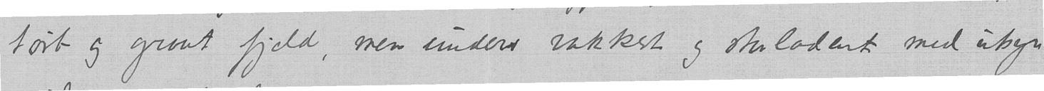tørt og graat fjeld, men unders vakkert og storladent med utsyn
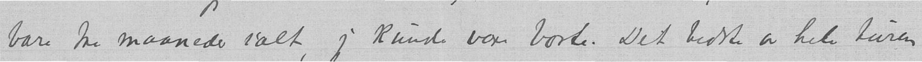bare tre maaneder ialt, jeg kunde være borte. Det bedste av hele turen
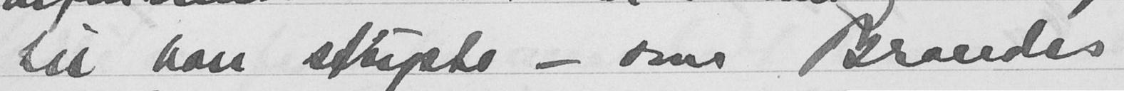til han stupte - som Brandes
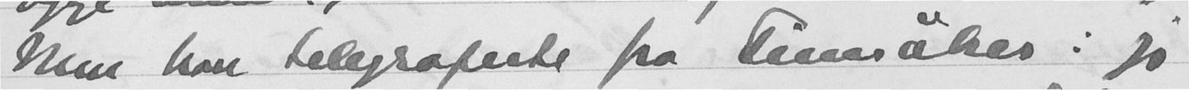Men han telegraferte fra Finnåker: jeg
